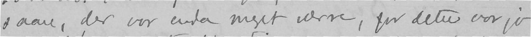saare, der var enda meget værre, for dette var jo
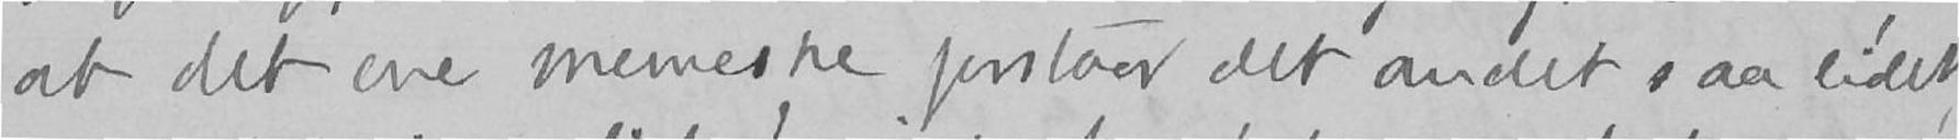at det ene menneske forstaar det andet saa lidet,
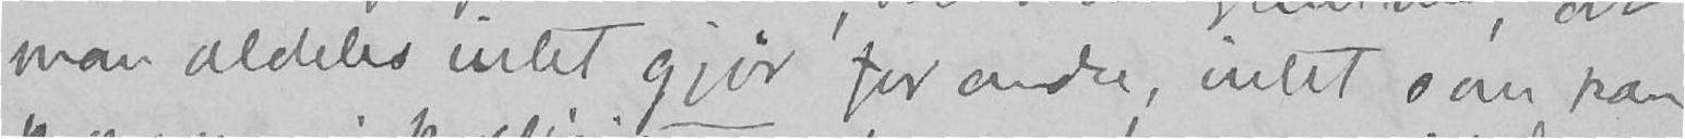man aldeles intet gjør for andre, intet som kan
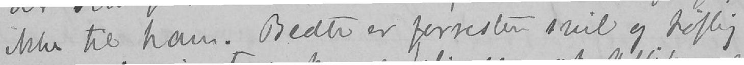ikke til ham. Beate er forresten snil og høflig
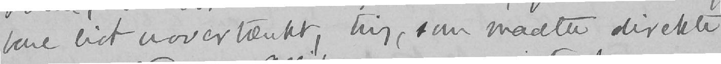bare lidt uovertænkt, ting, som maatte direkte
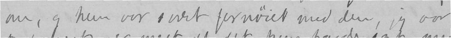om, og hun var svært fornøiet med den, jeg var
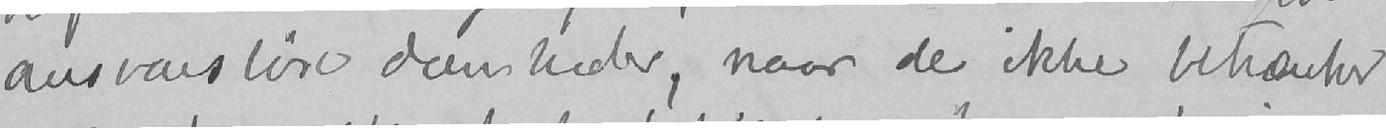ansvarsløse dumheder, naar de ikke betænker
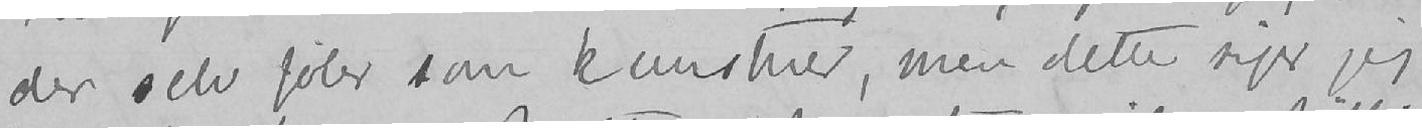der selv føler som kunstner, men dette siger jeg
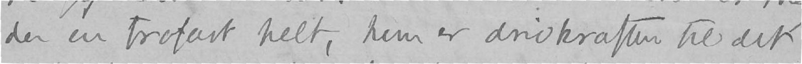da en trofast helt, hun er drivkraften til det
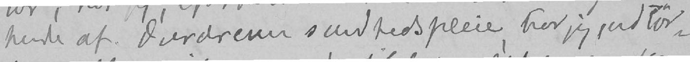hende af. Overdreven sundhedspleie, tror jeg, udtør-
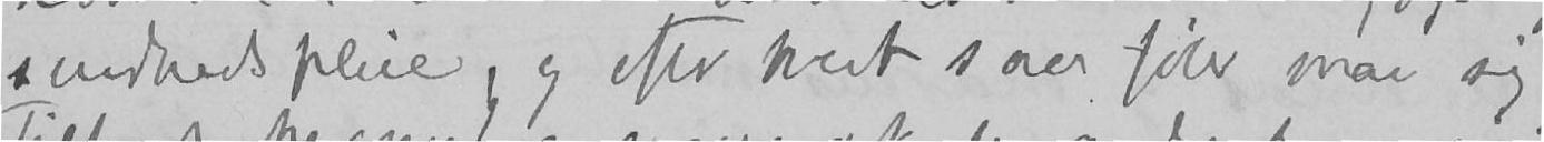sundhedspleie, og efter hvert saa føler man sig
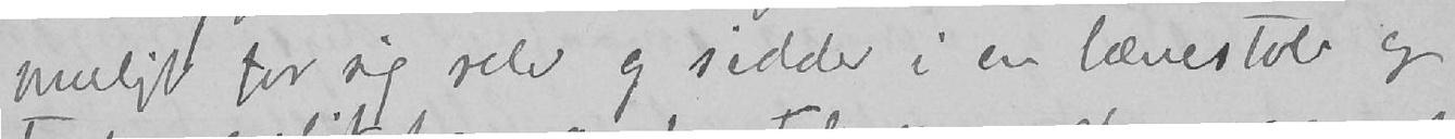muligt for sig selv og sidder i en lænestol og
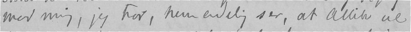med mig, jeg tror, hun endelig ser, at Allik vil
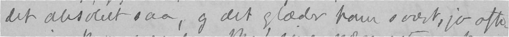det absolut saa, og det glæder ham svært, jo ofte-
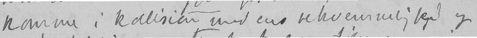komme i kollision med ens ubekvemmelighed og
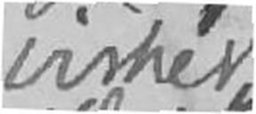virket
